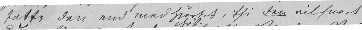fatte den end med Hjertet, thi den vil snart
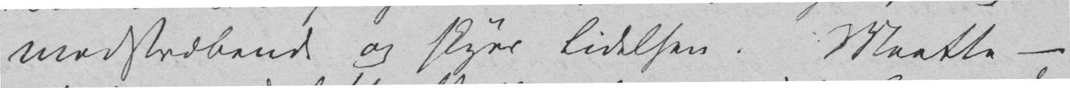modstræbende og skyer Lidelsen. Maatte –
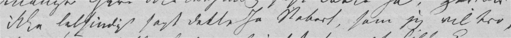ikke letsindigt sagt dette Ja Robert, som jeg vil tro,
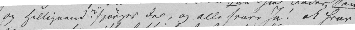og Helligaand?» spørges der, og alle svare Ja! ak hvor
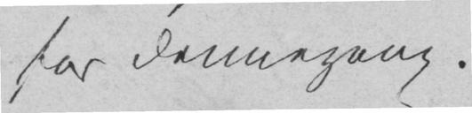for dennegang.
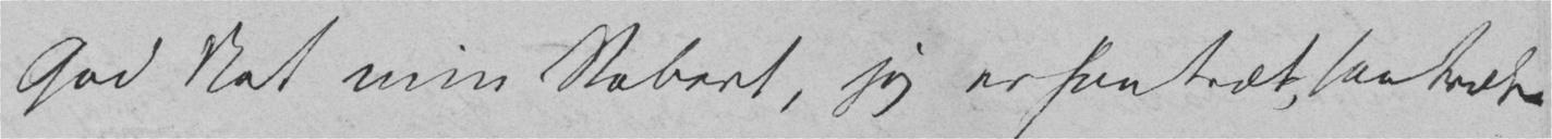God Nat min Robert, jeg er saa træt, saa træt.
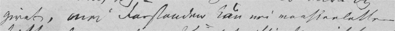givet, med Forstanden kan vi maaskee lettere
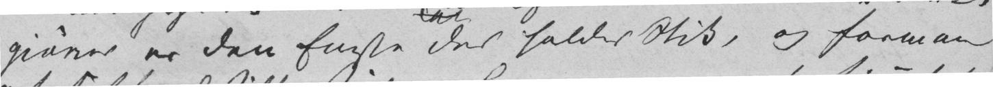er den Eneste der holder Stik, og formaa
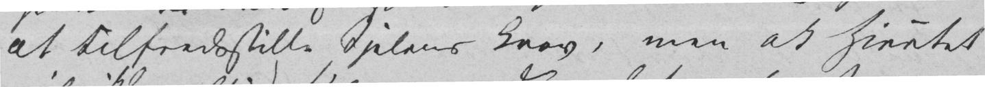at tilfredsstille Sjelens Krav, men ak Hjertet
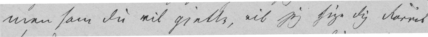men som Du vil gjette, vil jeg sige Dig Farvel
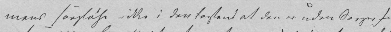mens sorgløse – ikke i den Forstand at den er uden Sorger –
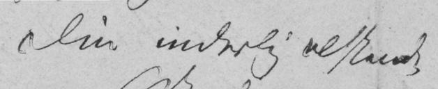Din inderlig elskende
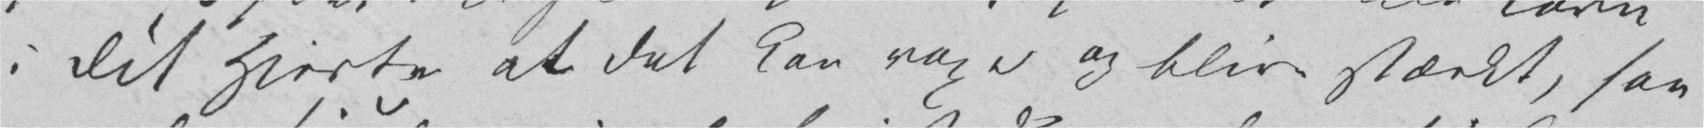i Dit Hjerte at det kan voxe og blive stærkt, saa
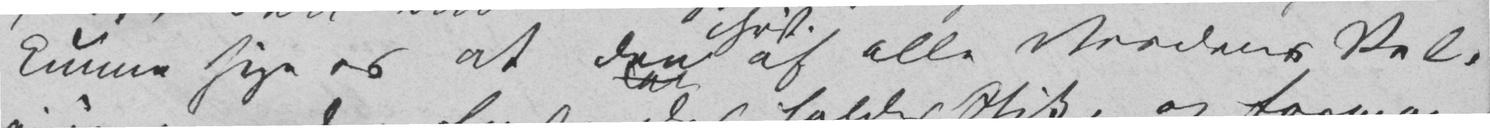kunne sige os at den af alle Verdens Religioner
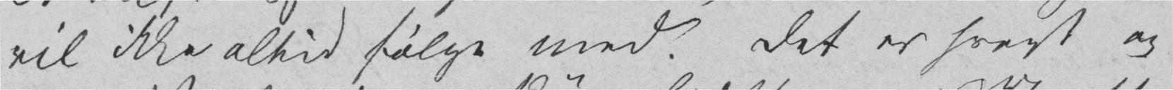vil ikke altid følge med. Det er svagt og
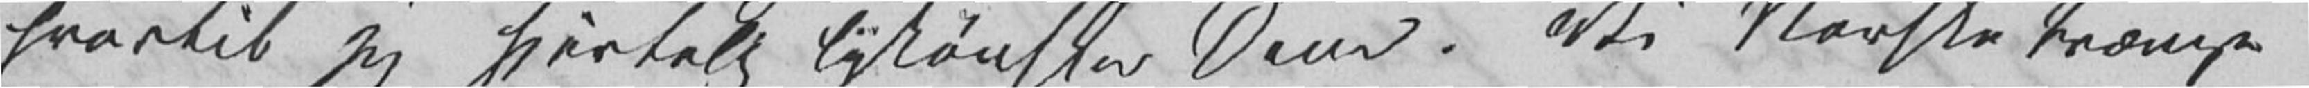hvortil jeg hjertelig lykønsker Dem. Vi Norske trænge
